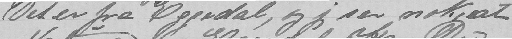Det er fra Eggedal, og jeg ser nok, at
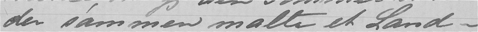der sammen malte et Land-
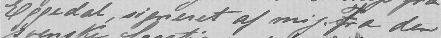Eggedal, signeret af mig. Fra den
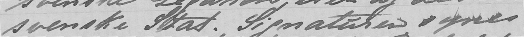svenske Stat. Signaturen synes
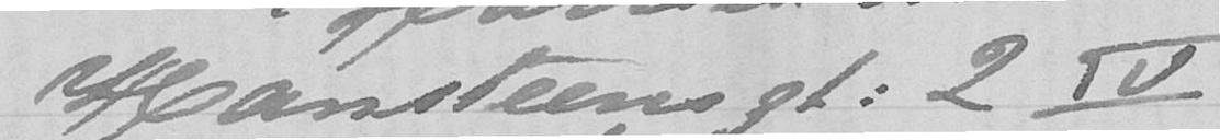Hansteensgt. 2 IV
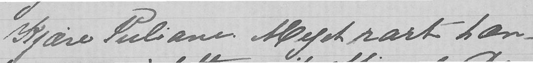Kjære Juliane. Meget rart hæn-
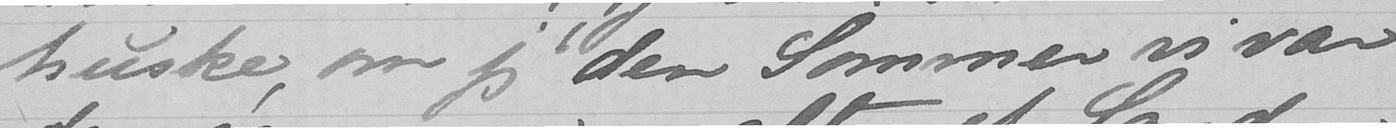huske, om jeg den Sommer vi var
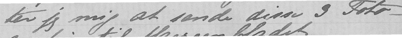ter jeg mig at sende disse 3 Foto-
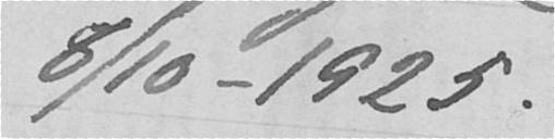8/10-1925.
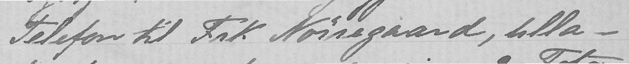Telefon til Frk Nørregaard, tilla-
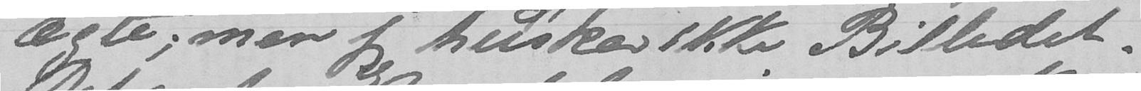ægte; men jeg husker ikke Billedet.
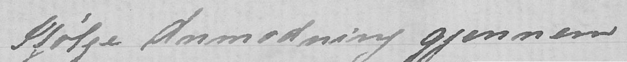Ifølge Anmodning gjennem
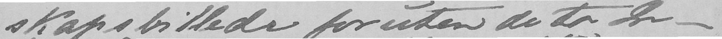skapsbillede foruten de to In-
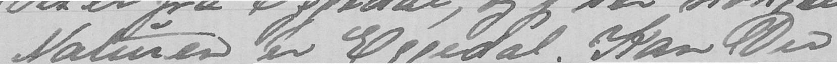Naturen er Eggedal. Kan Du
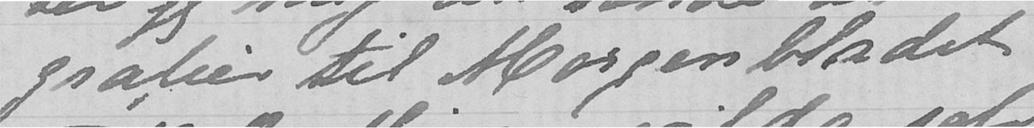grafier til Morgenbladet
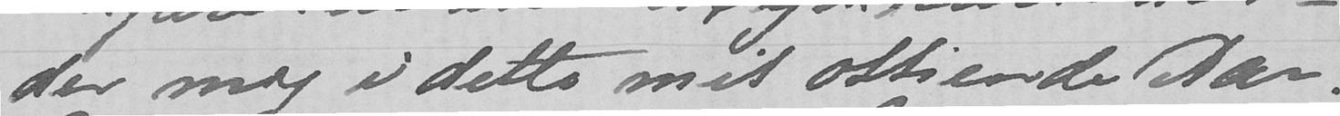der mig i dette mit ottiende Aar.
